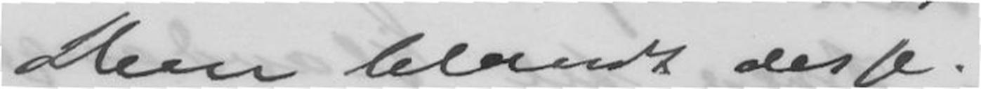Dem blandt desse.
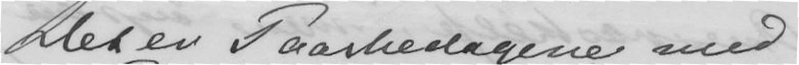Det er Paaskedagene med
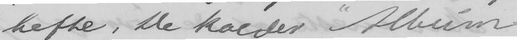hefte, De kalder "Album
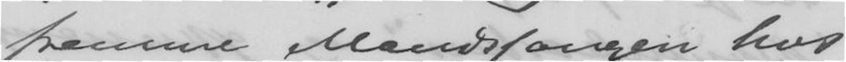fremme Manfssangen hos
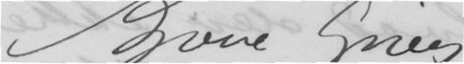Kjære Grieg
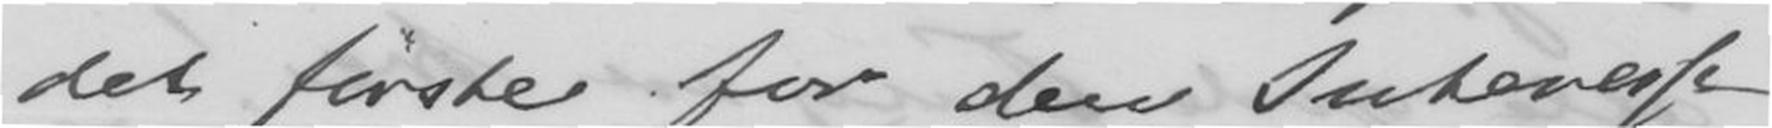det første for den Interesse
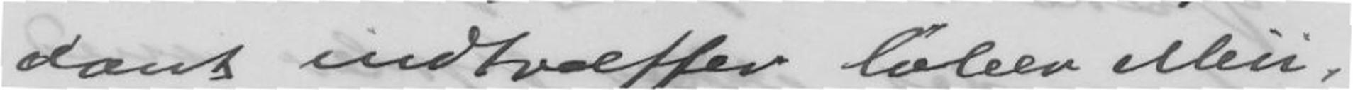dant indtræffer løber Min-
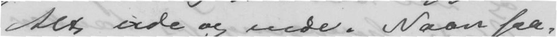Alt ude og inde. Naar saa-
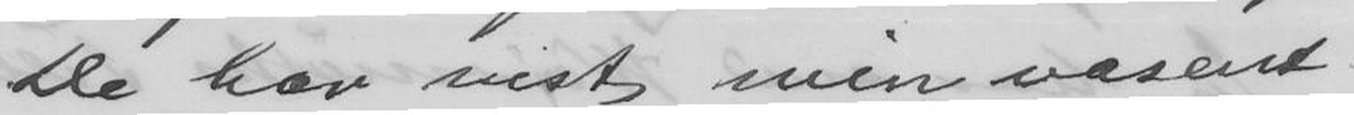De har vist min vesent-
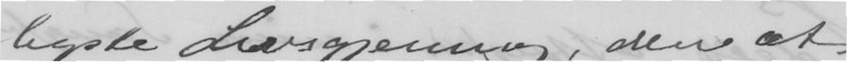ligste Livsgjerning, den at
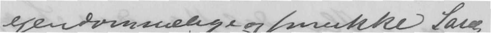ejendommelige og smukke Sang-
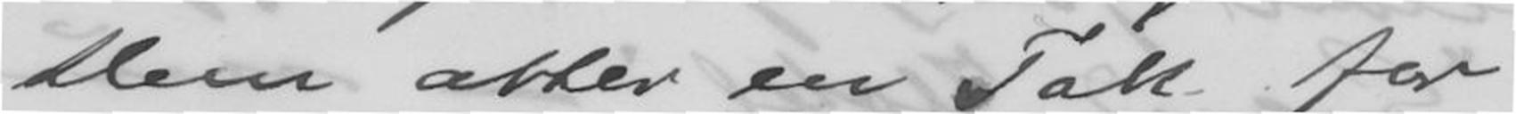Dem atter en Tak for
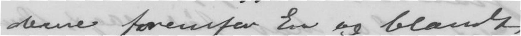derne fremfor En og blandt
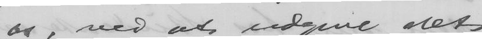os, ved at udgive det
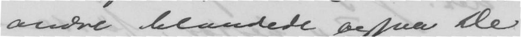andre blandede ogsaa De
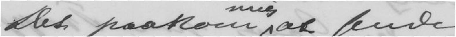Det paakom at sende
